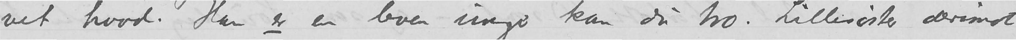vet hvad. Han er en brav unge kan du tro. Lillesøster derimot
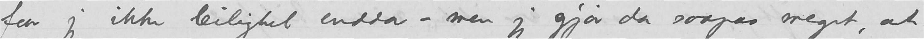faar jeg ikke leilighet endda -men jeg gjør da saapas meget, at
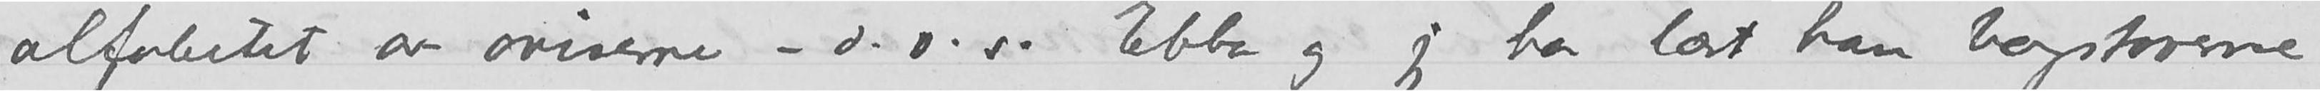alfabetet av aviserne - d.v.s. Ebba og jeg har lært ham bogstaverne
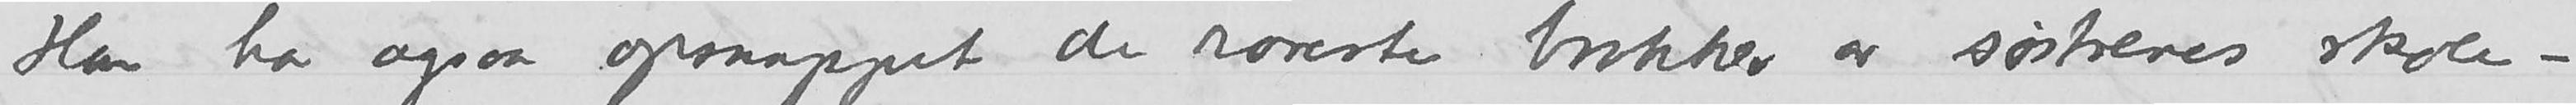Han har ogsaa opsnappet de rareste brokker av søstrenes skole-
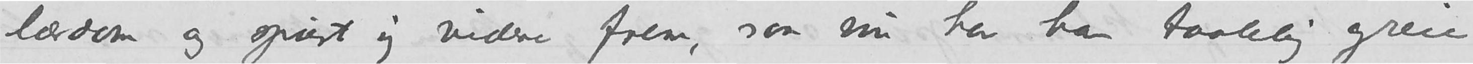lærdom og spurt sig videre frem, saa nu har han taalelig greie
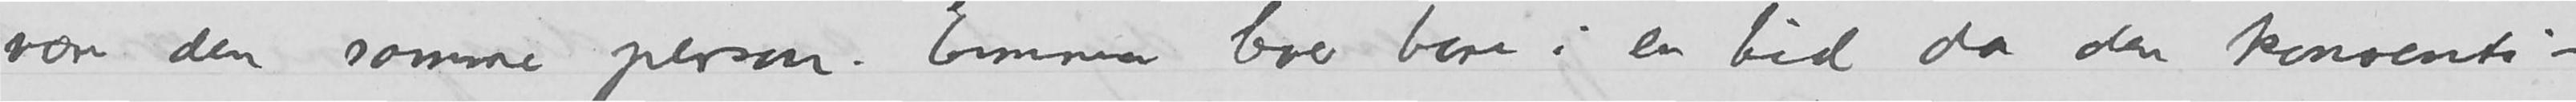være den samme person. Emma lever bare i en tid da den konventio
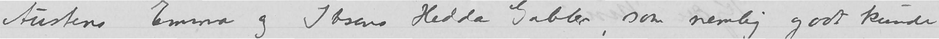Austens Emma og Ibsens Hedda Gabler, som nemlig godt kunde
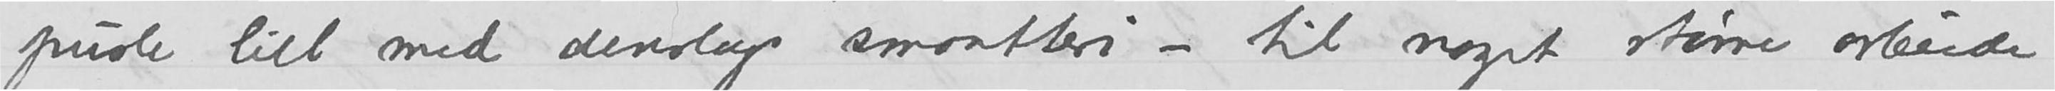pusle litdt med  denslags smaatteri -til noget større arbeide
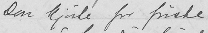Don kjørte for første
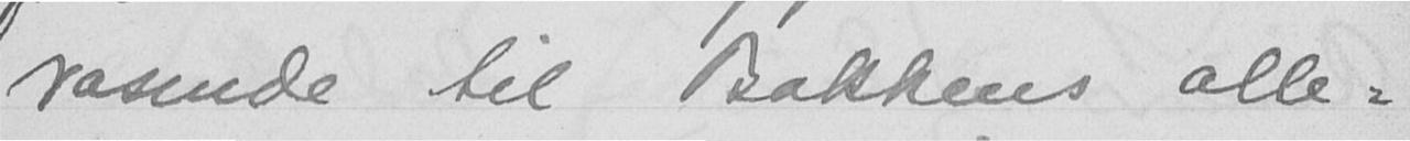rasende til Bokkens alle-
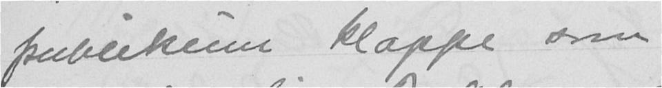publikum klappe som
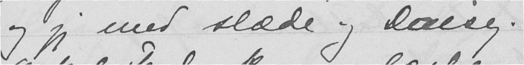og jeg med slæde og Dansig.
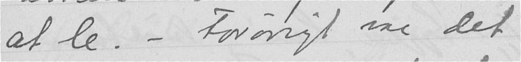at le. - Forøvrigt var det
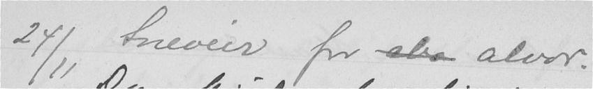24/2 Sneveir for abs alvor.
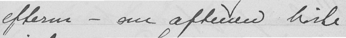efterm - om aftenen hørte
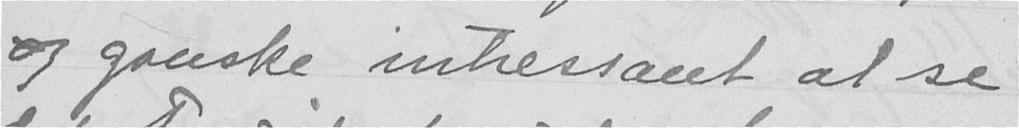og ganske intressant at se
